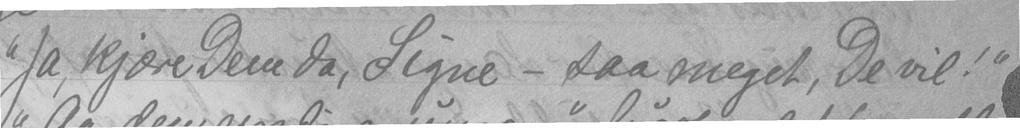"Ja, kjære Dem da, Signe - saa meget, De vil!"
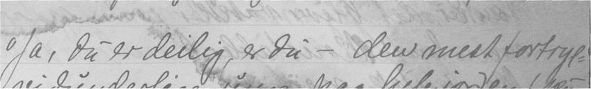"Ja, du er deilig, er du - den mest fortryl-
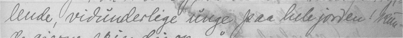lende, vidunderlige unge paa hele jorden! kun-
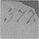377.
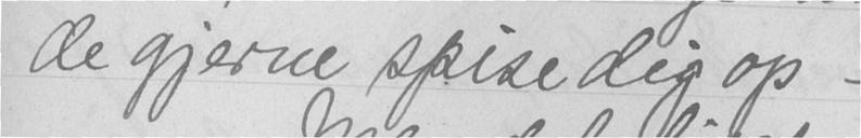de gjerne spise dig op -"
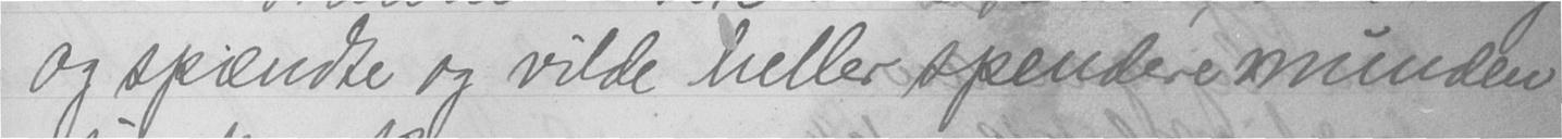og spændte og vilde heller spendere munden
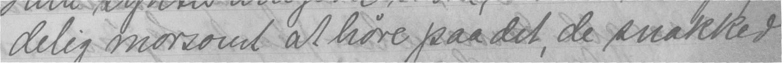deilig morsomt at høre paa det, de snakked
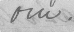om.
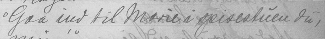"Gaa ind til Marie i spisestuen du,
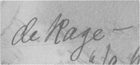de kage -
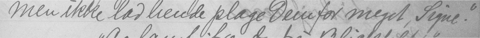men ikke lad hende plage Dem for meget, Signe."
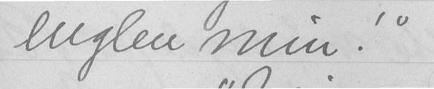englen min!"
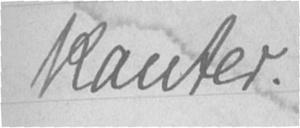kanter.
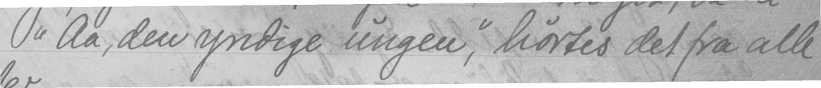"Aa, den yndige ungen," hørtes det fra alle
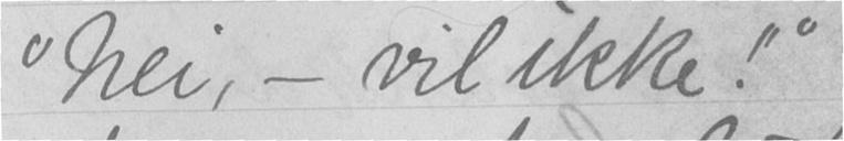"Nei, - vil ikke!"
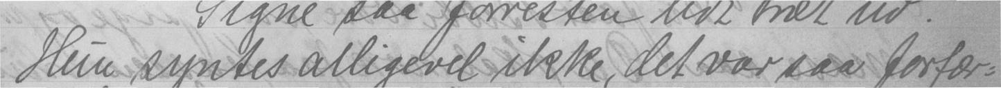Hun syntes alligevel ikke, det var saa forfær-
